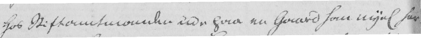Hos Stiftamtmanden ude paa en Gaard han nyel har
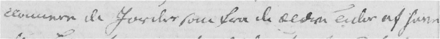clamere de Jorder som fra de ældre Tider af have
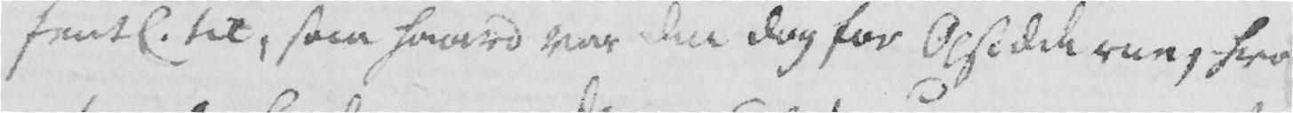fentl. til, saa haard var den dog for Opsidderne, hvor-
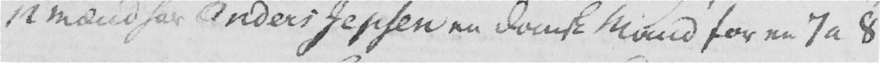12 Mænd har Anders Jepsen en dansk Mand for en 7 a 8
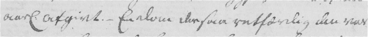aarl. afgivt. - En Dom der saa retfærdig den var
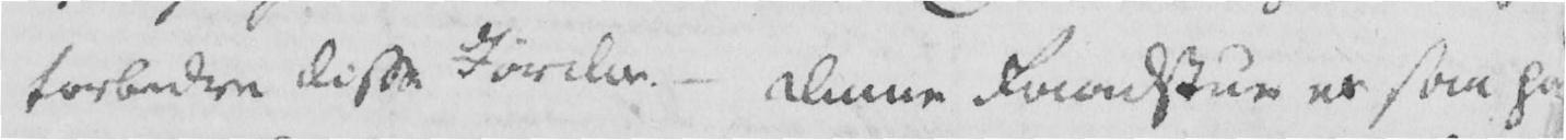forbedre disse Jorder. - Denne Raadstue er som paa
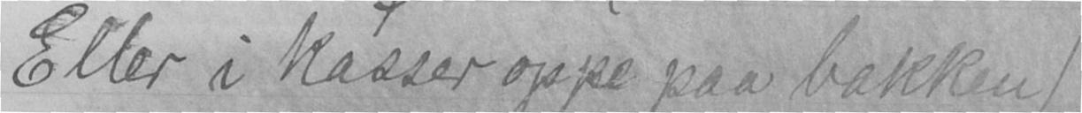Eller i kasser oppe paa bakken)
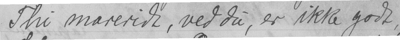Thi mareridt, ved du, er ikke godt,
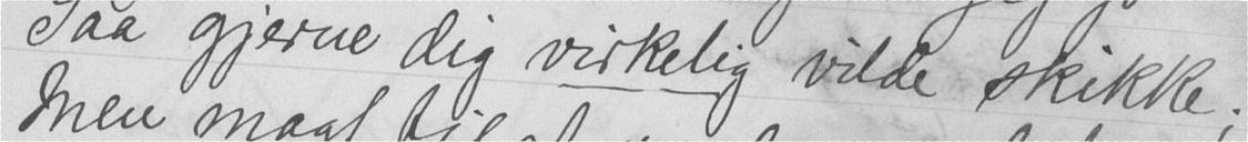Saa gjerne dig virkelig vilde skikke;
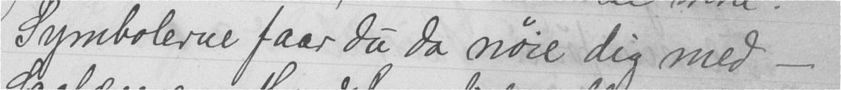Symbolerne faar du da nøie dig med -
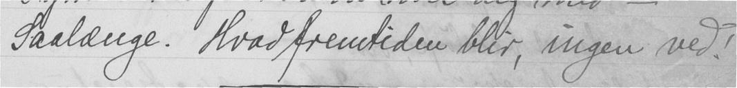Saalænge. Hvad fremtiden blir, ingen ved!"
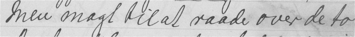men magt til at raade over de to
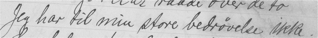Jeg har til min store bedrøvelse ikke.
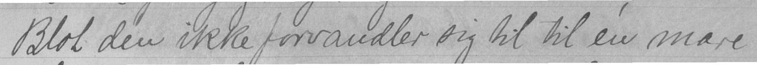Blot den ikke forvandler sig til til en mare!
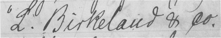"L. Birkeland & co.
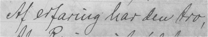Af erfaring har den tro,
